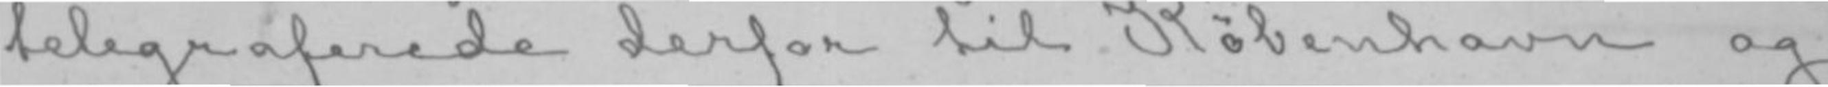telegraferede derfor til København og
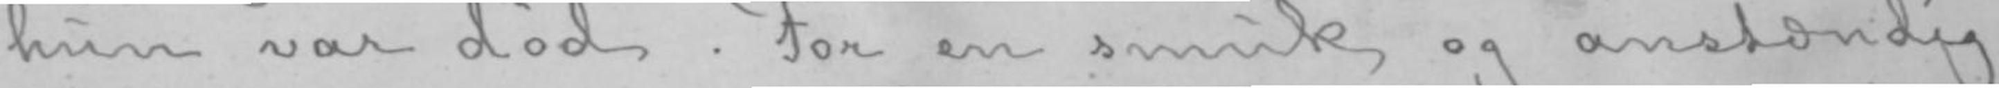hun var død. For en smuk og anstændig
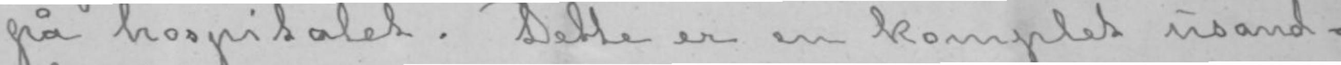på hospitalet. Dette er en komplet usand-
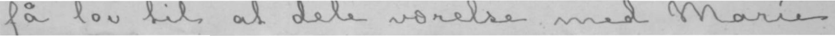få lov til at dele værelse med Marie,
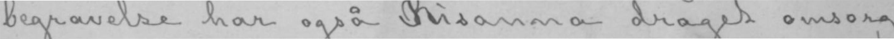begravelse har også Susanna draget omsorg,
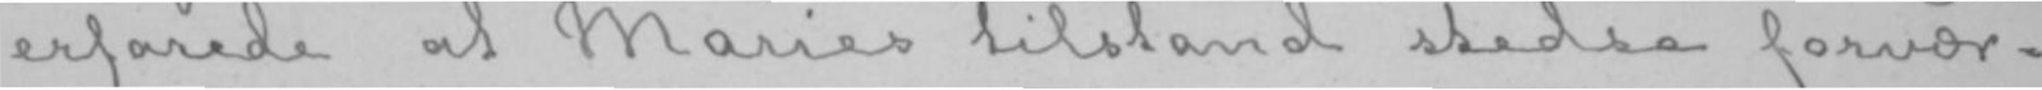erfarede at Maries tilstand stedse forvær-
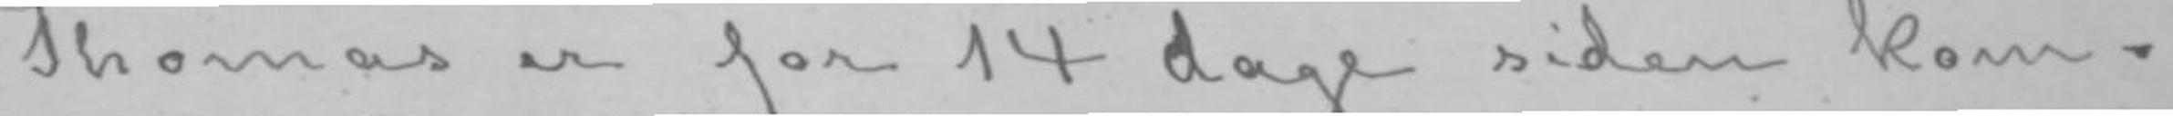Thomas er for 14 dage siden kom-
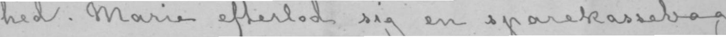hed. Marie efterlod sig en sparekassebog,
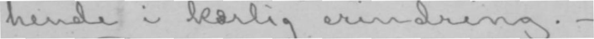hende i kærlig erindring.-
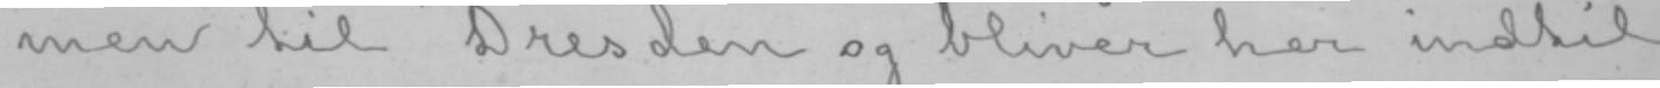men til Dresden og bliver her indtil
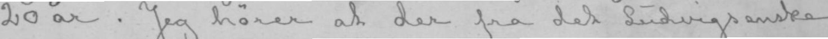20 år. Jeg hører at der fra det Ludvigsenske
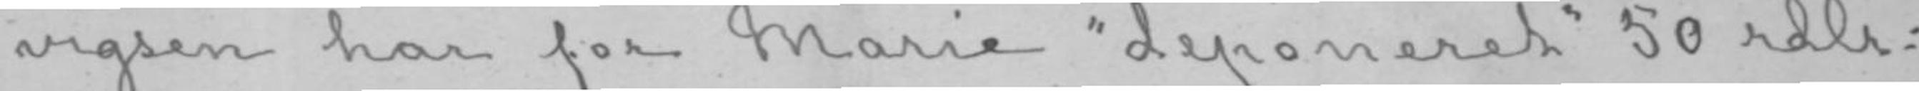vigsen har for Marie «deponeret» 50 rdlr:
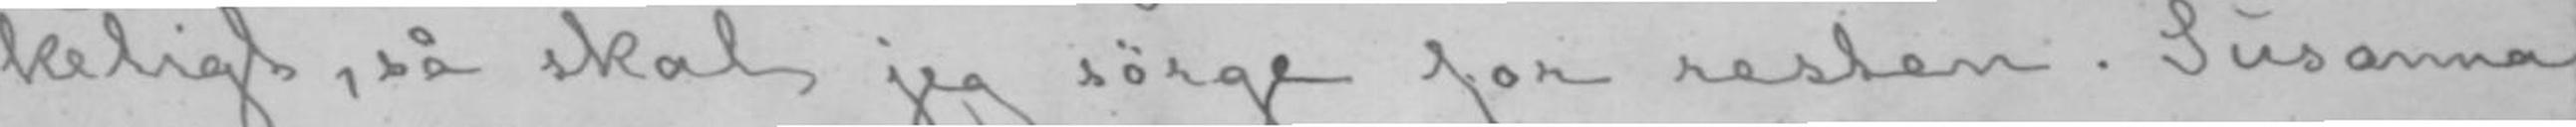keligt, så skal jeg sørge for resten. Susanna
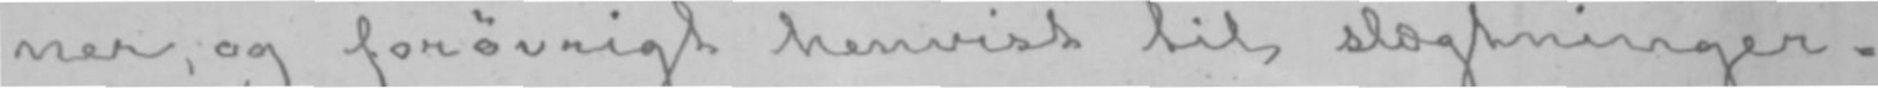ner, og forøvrigt henvist til slægtninger-
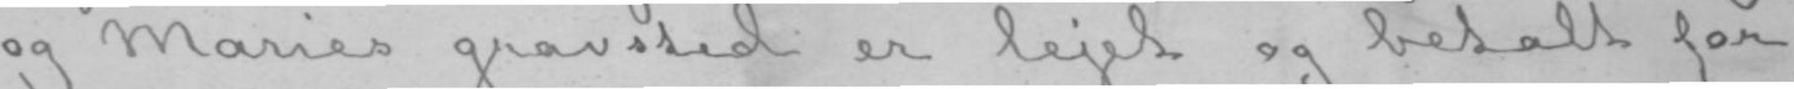og Maries gravsted er lejet og betalt for
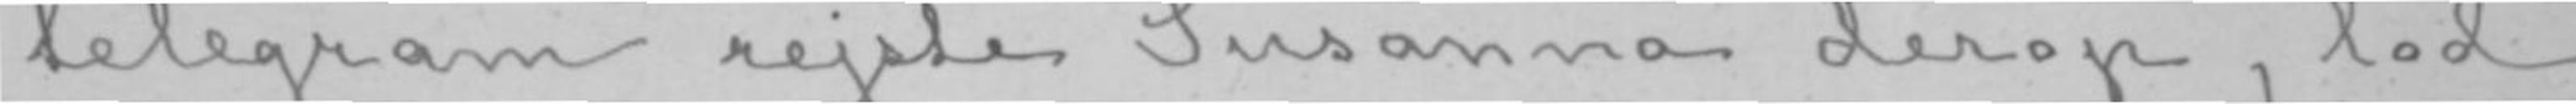telegram rejste Susanna derop, lod
